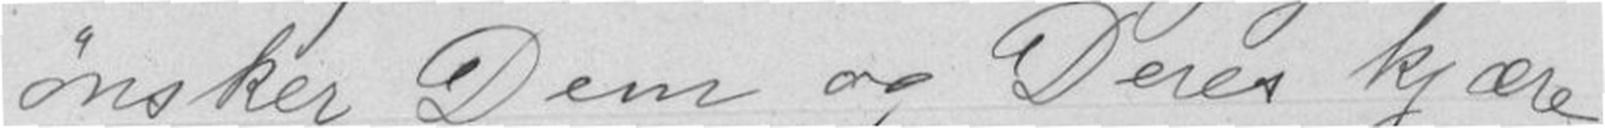ønsker Dem og Deres kjære
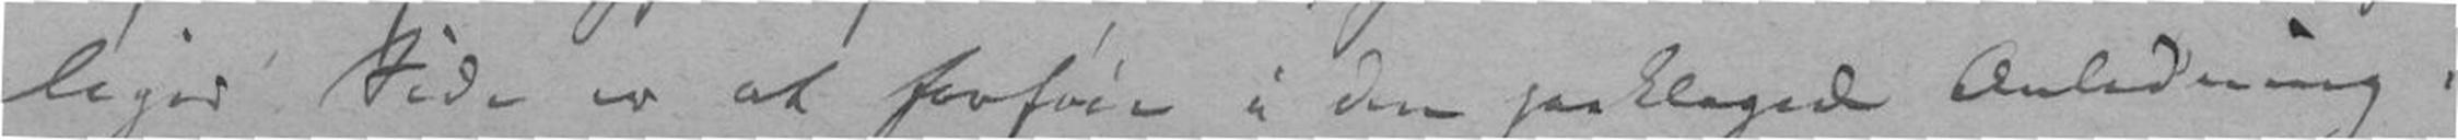liges sider er at forføie i den paaklagede Anledning.
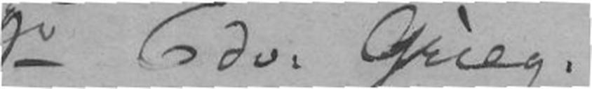For. Edv. Grieg.
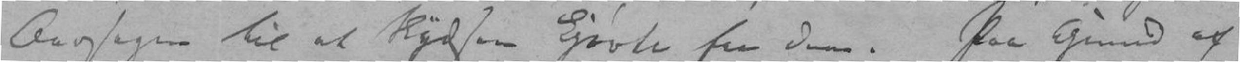til at skydse for dem. Paa Grund af
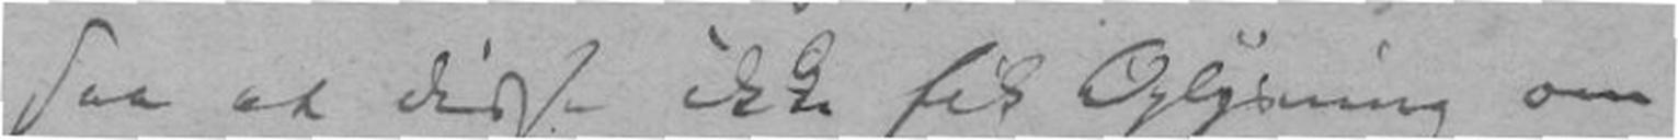saa at sligt ikke fik Oplysning om
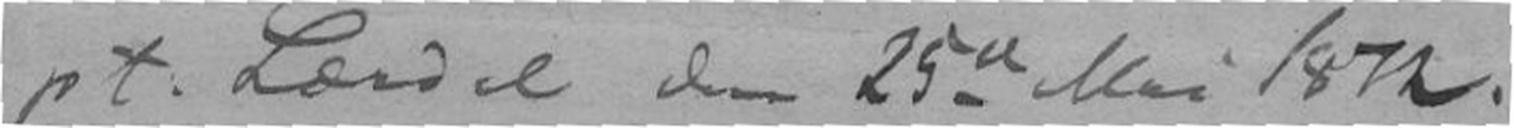pt. deb 25 Mai 1872
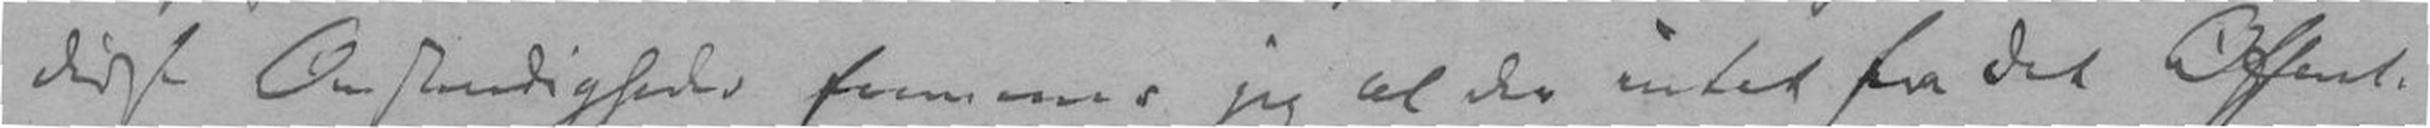disse Omstændigheder jeg at der intet fra det Offent-
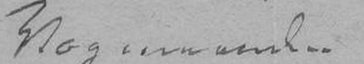Vognmanden
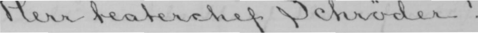Herr teaterchef Schrøder!
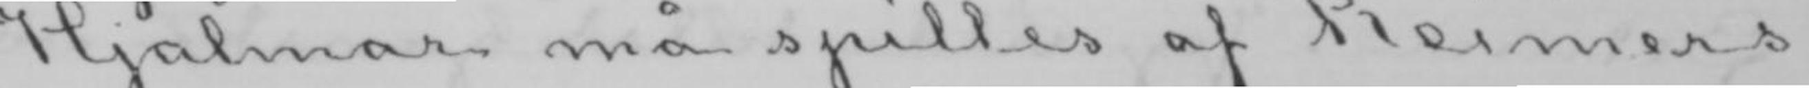Hjalmar må spilles af Reimers.
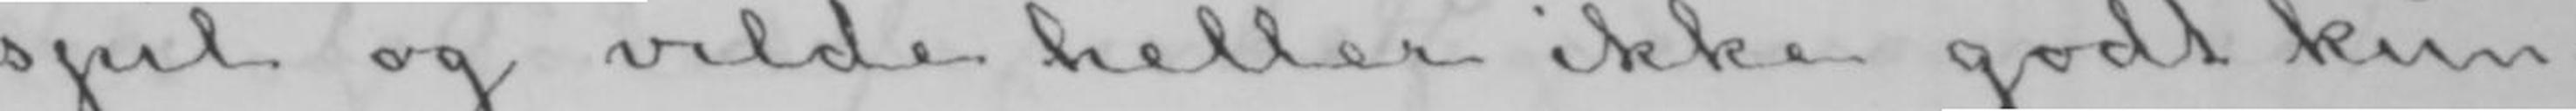spil og vilde heller ikke godt kun-
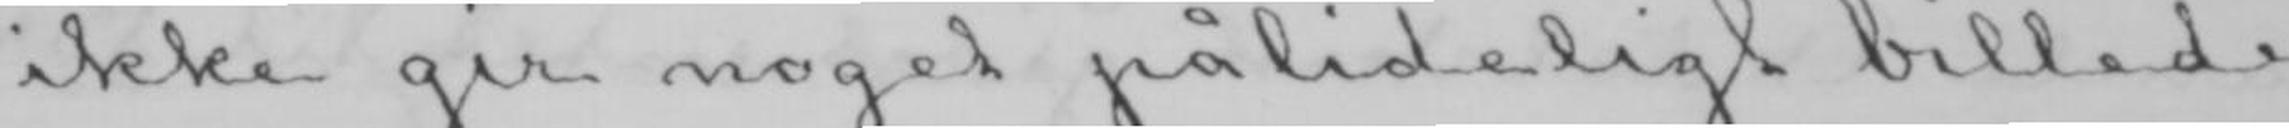ikke gir noget pålideligt billede.
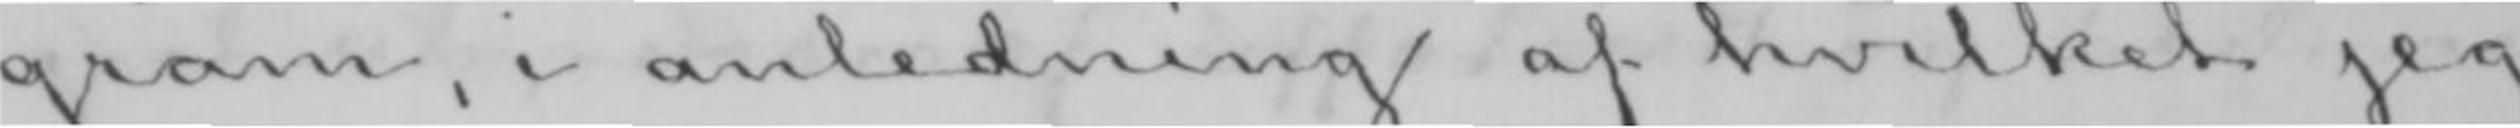gram, i anledning af hvilket jeg
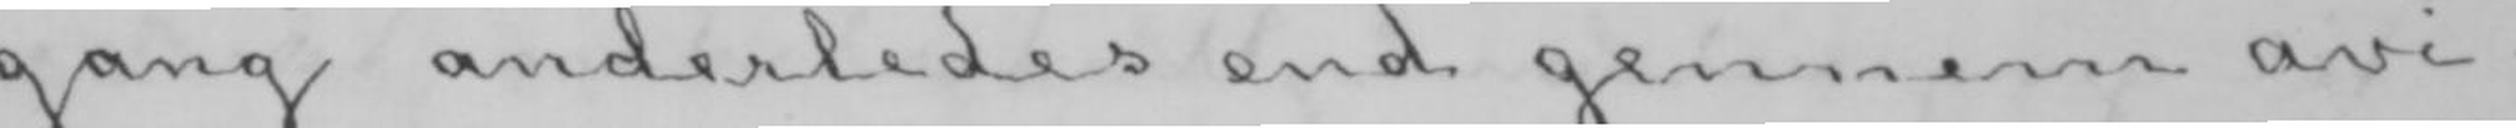gang anderledes end gennem avi-
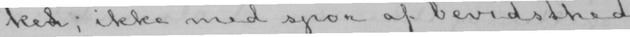kent; ikke med spor af bevidsthed
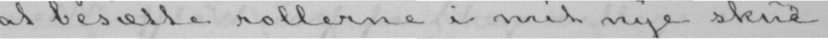at besætte rollerne i mit nye skule-
